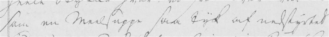som en Meelsuppe saa tyk af nedstyrtet
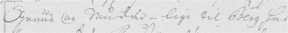Gruus og Mudder - lige til Berg har
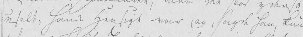uselt, hans Hensigt var og, sagde han, kun
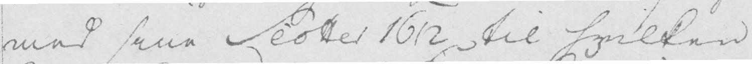med sine Scotter 1612 til hvilken
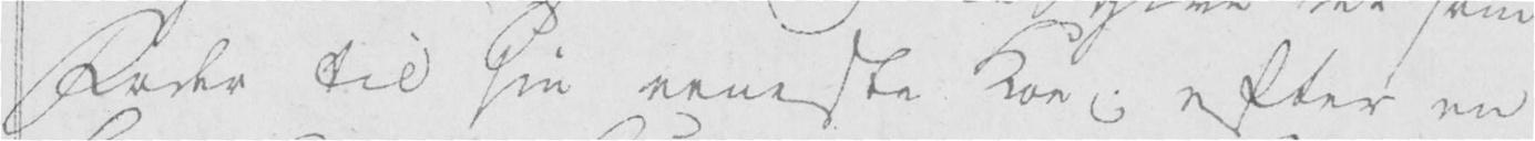Fader til sin eeneste Kon; efter en
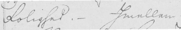Rolighed. Imellem
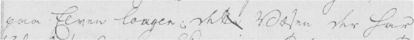paa Elven Laugen; dette Væsen der har
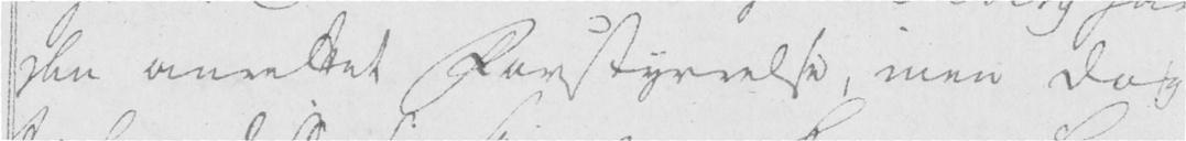den anrettet Forstyrrelse, men dog
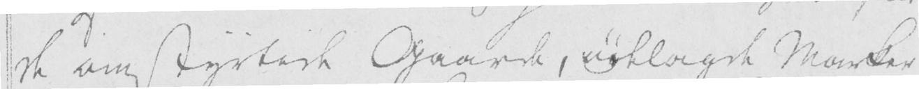de omstyrtede Gaarde, ødelagde Marker
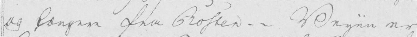og længere fra Rosten. - Veyen er
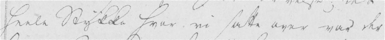heele Stykke hvor vi satte over var der
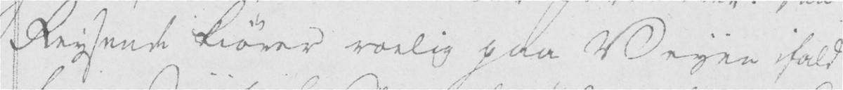Reysende kiører roelig paa Veyen ifald
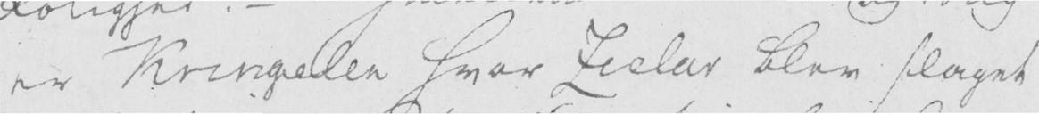er Kringelen hvor Ziclar blev slaget
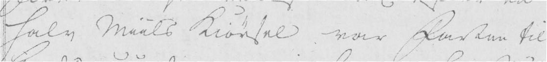halv Miils Kiørsel var Farten til
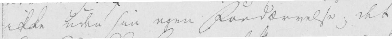ikke uden sin egen Fordærvelse; det
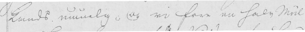Lands umuelig; og vi fore en halv Miil
